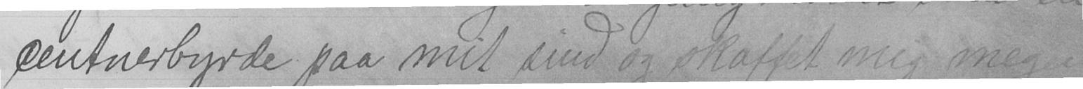centnerbyrde paa mit sind og skaffet mig meget
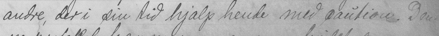andre, der i sin tid hjalp hende med caution. Den-
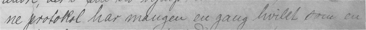ne protokol har mangen en gang hvilet som en
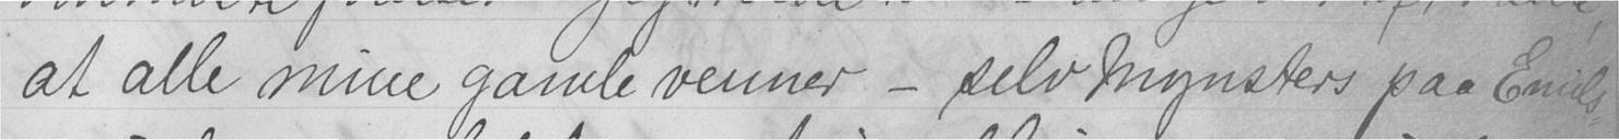at alle mine gamle venner - selv Mynsters paa Emils-
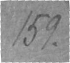159.
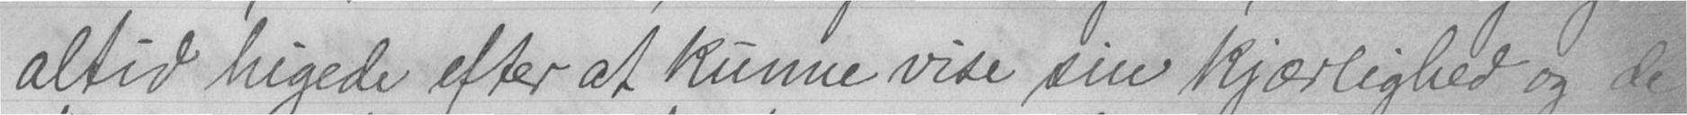altid higede efter at kunne vise sin kjærlighed og de
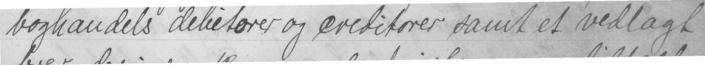boghandels debitorer og creditorer samt et vedlagt
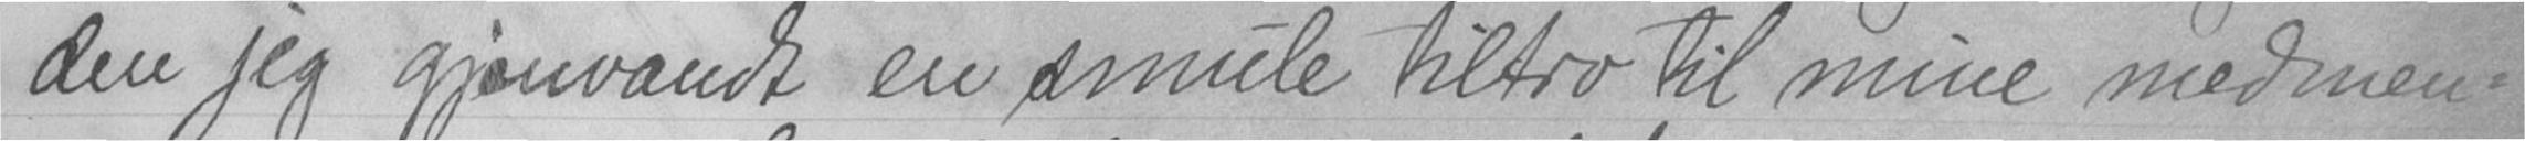den jeg gjenvandt en smule tiltro til mine medmen-
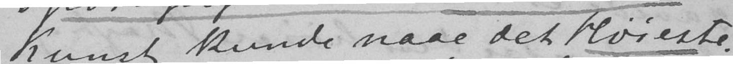Kunst kunde naae det Høieste.
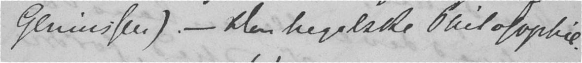Glassen). – Den hegelske Philosophie.
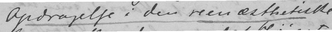Opdragelse i den reen æsthetiske
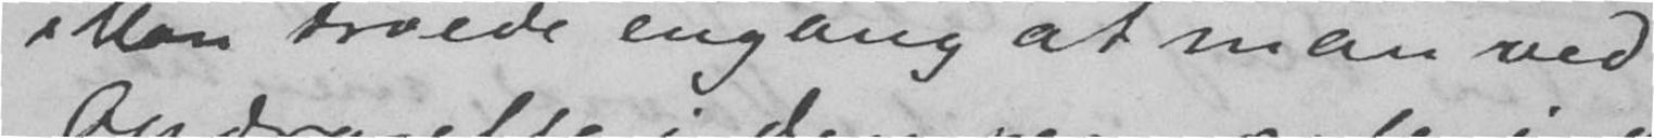Man troede engang at man ved
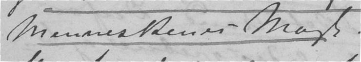Menneskens Magt.
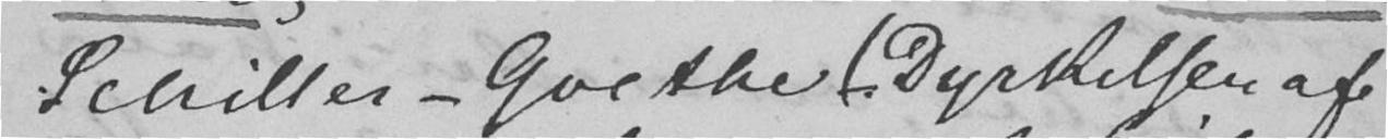Schiller – Goethe (Dyrkelsen af
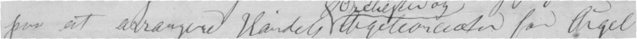paa at arrangere Händels orgelconcerter for Orgel
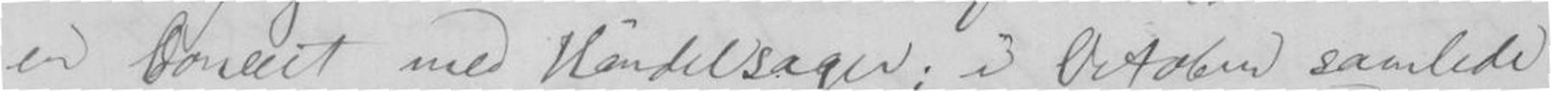en Concert med Händelsager; 3 October samlede
